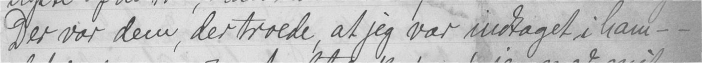Der var dem, der troede at jeg var indtaget i ham - -
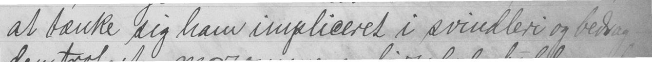at tænke sig ham impliceret i svindleri og bedrag -
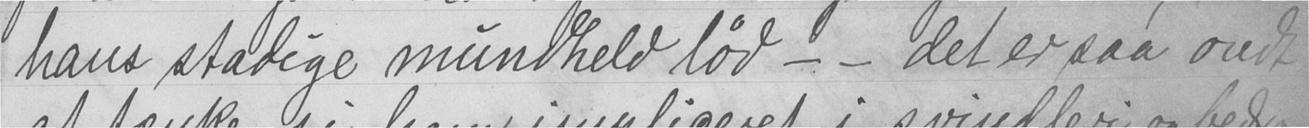hans stadige mundheld lød - - det er saa ondt
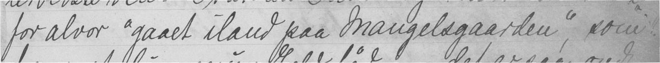for alvor "gaaet iland paa Mangelsgaarden", som
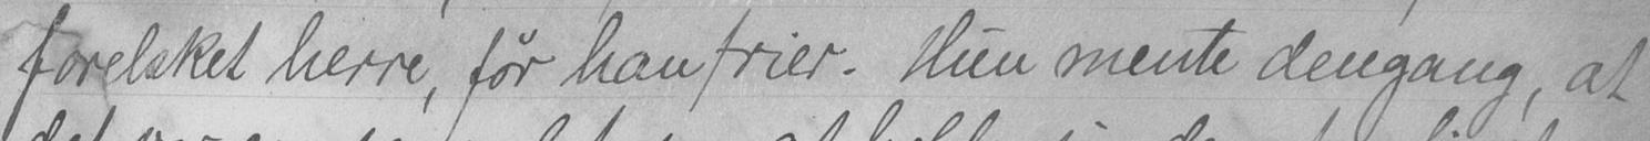forelsket herre, før han frier. Hun mente dengang, at
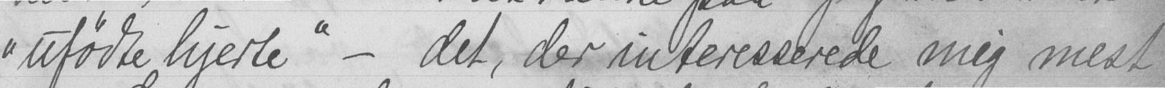"ufødte hjerte" - det, der interesserede mig mest
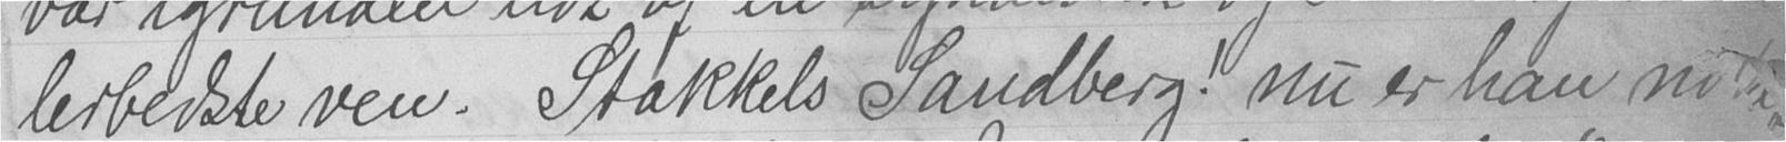lerbedste ven. Stakkels Sandberg! nu er han nok
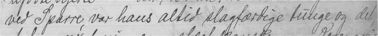ved Sparre, var hans altid slagfærdige tunge og det,
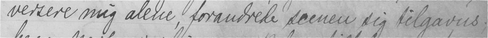versere mig alene, forandrede scenen sig tilgavns;
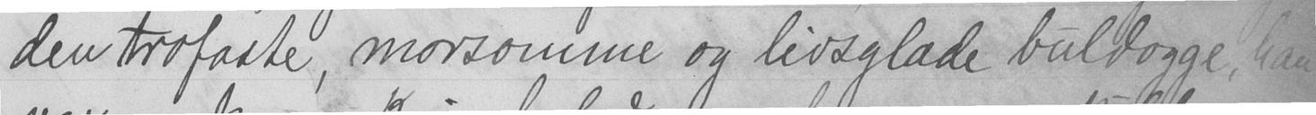den trofaste, morsomme og livsglade buldogge, han
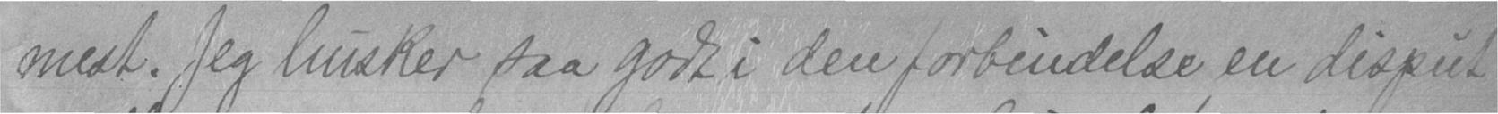mest. Jeg husker saa godt i den forbindelse en disput
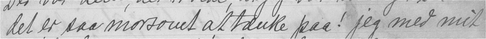det er saa morsomt at tænke paa! jeg med mit
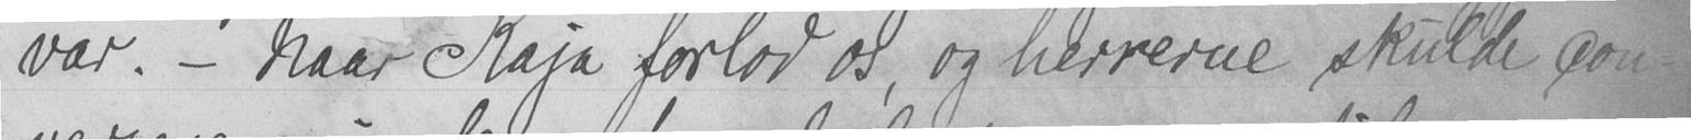var. - Naar Kaja forlod os, og herrerne skulde con-
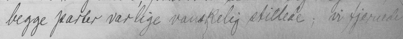begge parter var lige vanskelig stillede; vi fjernede
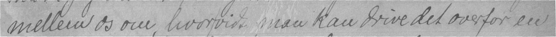mellem os om, hvorvidt man kan drive det overfor en
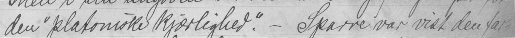den "platoniske kjærlighed." - Sparre var vist den far-
